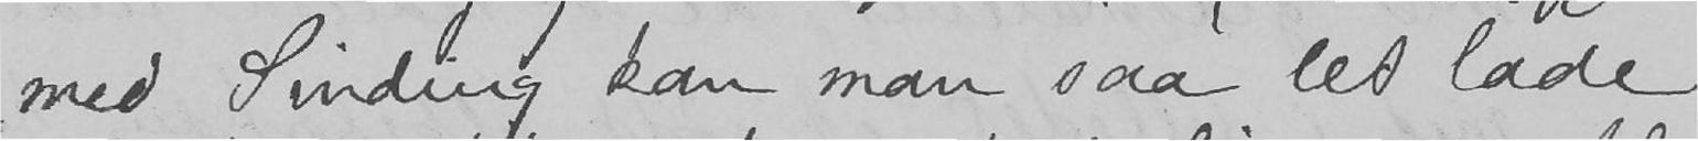med Sinding kan man saa let lade
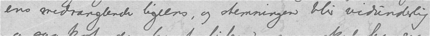ens medranglende ligeens, og stemningen blir vidunderlig
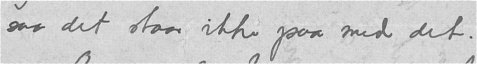saa det staar ikke paa med det.
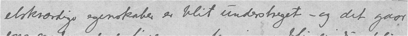elskværdige egenskaber er blit understreget – og det gaar
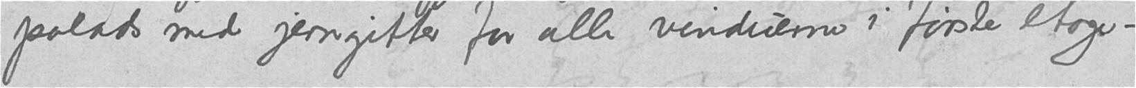palads med jerngitter for alle vinduerne i første etage –
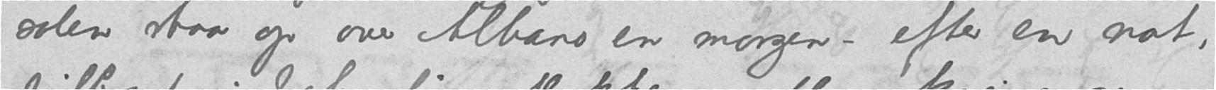solen staa op over Albans en morgen – efter en nat,
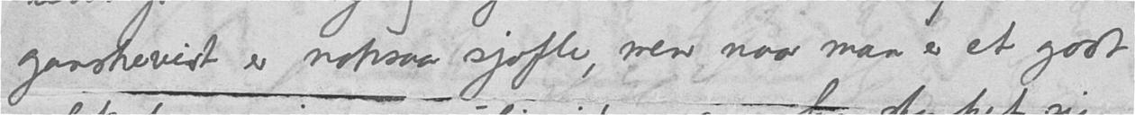ganskevist er noksaa sjofle, men naar man er et godt
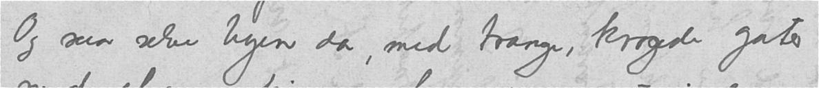Og saa selve byen da, med trange, krogede gater
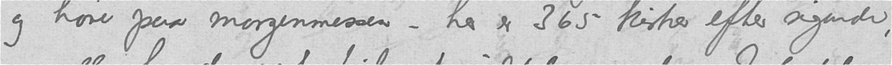og høre paa morgenmessen – her er 365 kirker efter sigende,
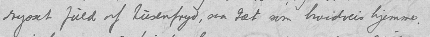drysset fuld af tusenfryd, saa tæt som hvidveis hjemme,
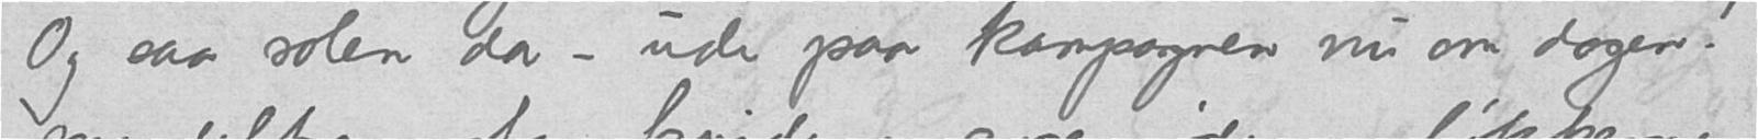Og saa solen da – ude paa kampagnen nu om dagen!
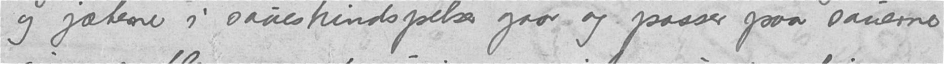og jæterne i saueskindspelser gaar og passer paa sauerne
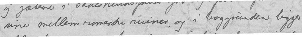sine mellem romerske ruiner, og i baggrunden ligger
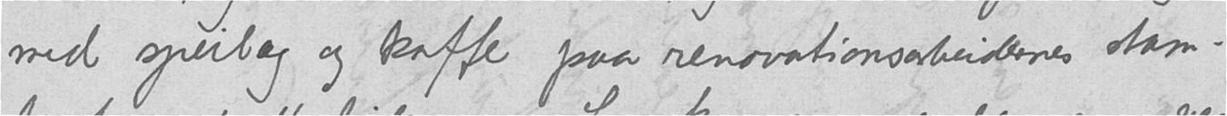med speilæg og kaffe paa renovationsarbeidernes stam-
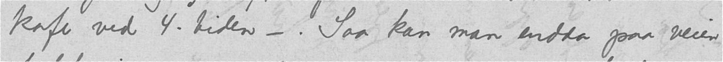kafe ved 4-tiden –. Saa kan man endda paa veien
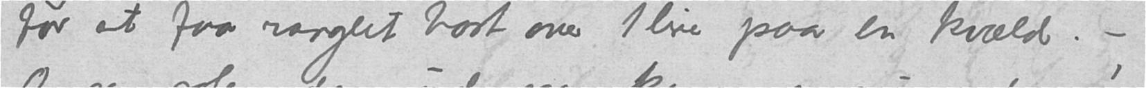for at faa ranglet bort over 1lire paa en kvæld.  –
